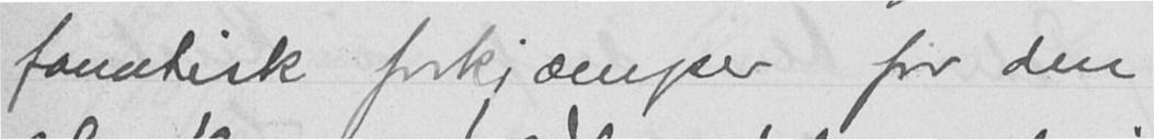fanatisk forkjæmper for den
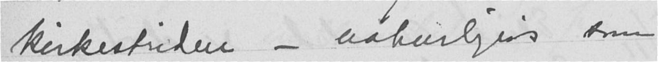kirkestriden - naturligvis som
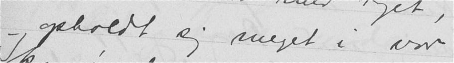og opholdt sig meget i vor
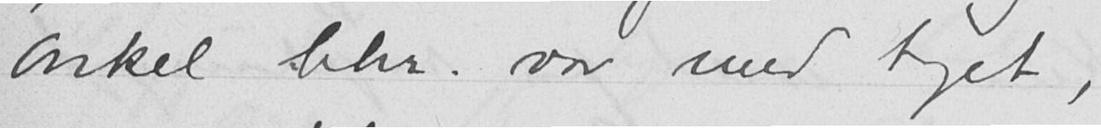Onkel Chr. var med toget,
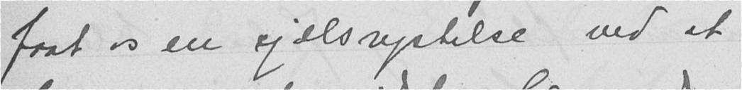faat os en sjælsrystelse ved at
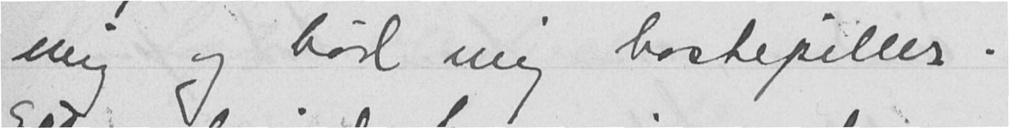mig og bød mig hostepiller.
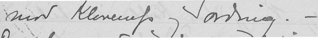mod Klaveness og Ording. -
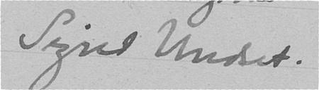Sigrid Undset.
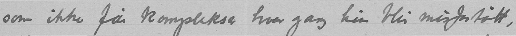som ikke får komplekser hver gang hun blir misforstått,
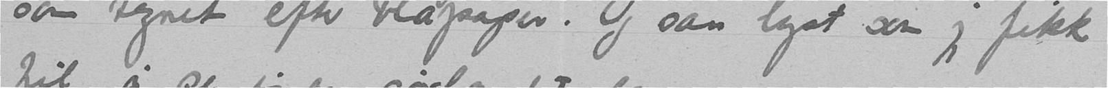som tegnet efter blåpapir. Og sån lyst som jeg fikk
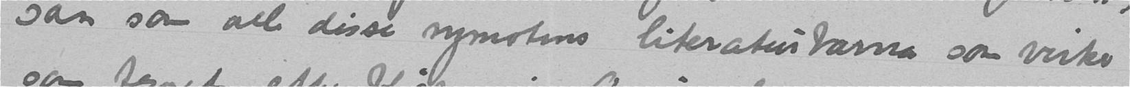sån som alle disse nymotens literaturbarna som virker
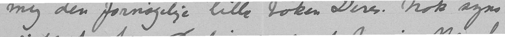mig den fornøyelige lille boken Deres. Nok syns
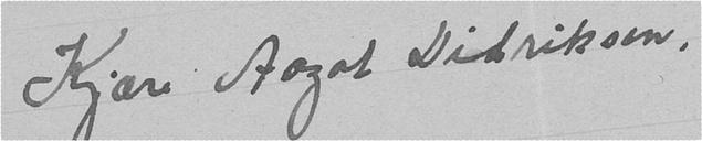Kjære Aagot Didriksen,
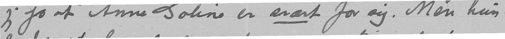jeg jo at Anne Goline er svært for sig. Men hun
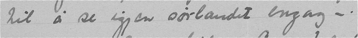til å se igjen sørlandet engang –.
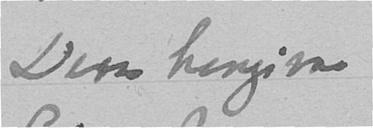Deres hengivne
